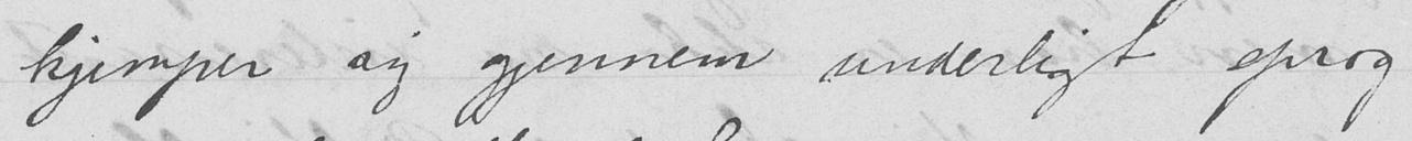kjemper sig gjennem underligt sprog
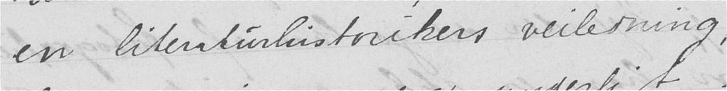en literaturhistorikers veiledning,
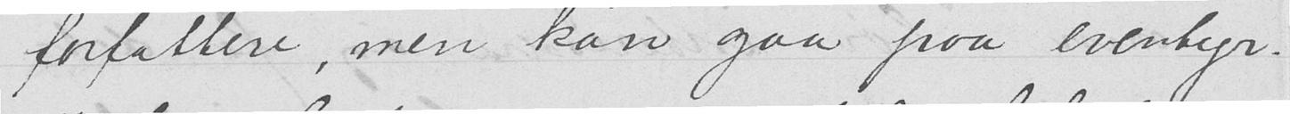forfattere, men kan gaa paa eventyr.
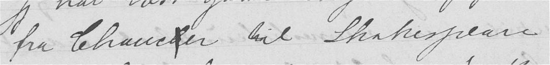fra Chaucler til Shakespeare
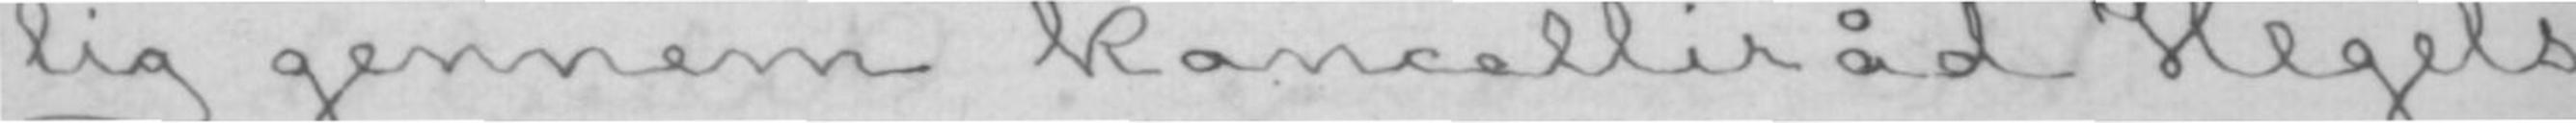lig gennem kancelliråd Hegels
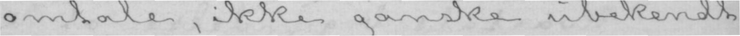omtale, ikke ganske ubekendt
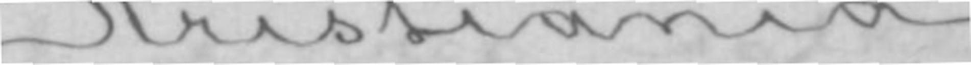Kristiania.
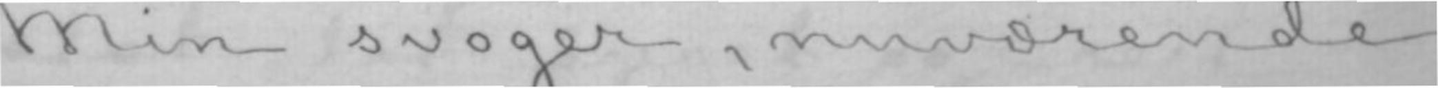Min svoger, nuværende
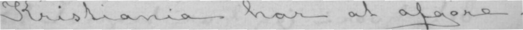Kristiania har at afgøre.
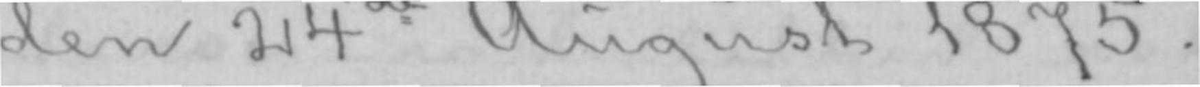den 24de August 1875.
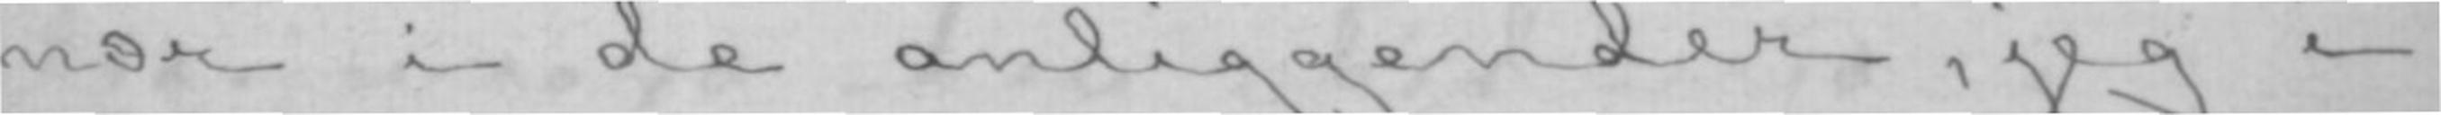nær i de anliggender, jeg i
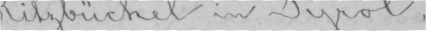Kitzbüchel in Tyrol,
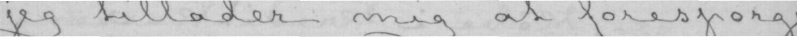jeg tillader mig at forespørge
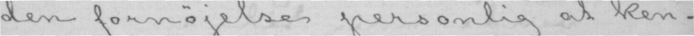den fornøjelse personlig at ken-
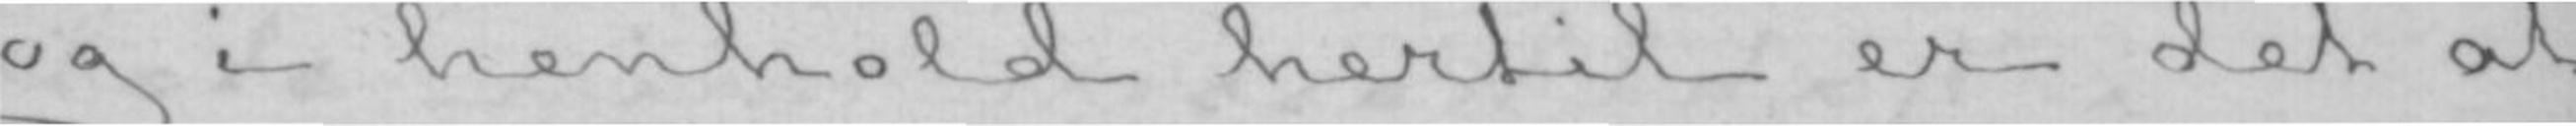og i henhold hertil er det at
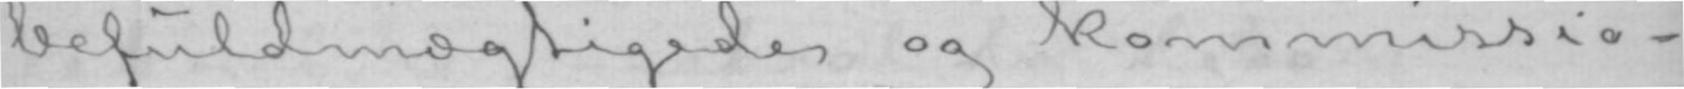befuldmægtigede og kommissio-
